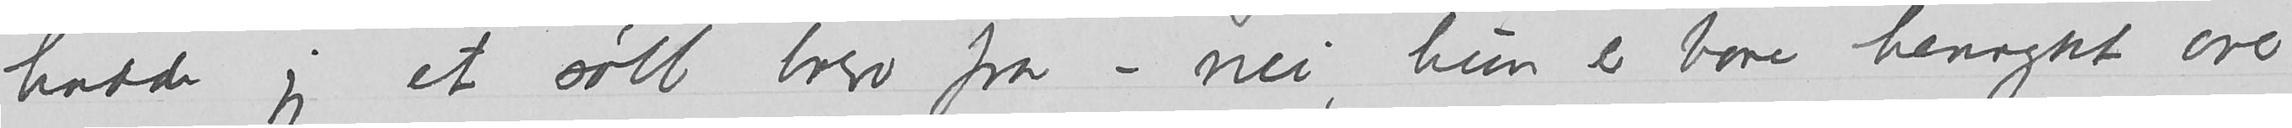hadde jeg et søtt brev fra – nei, hun er bare henrykt over
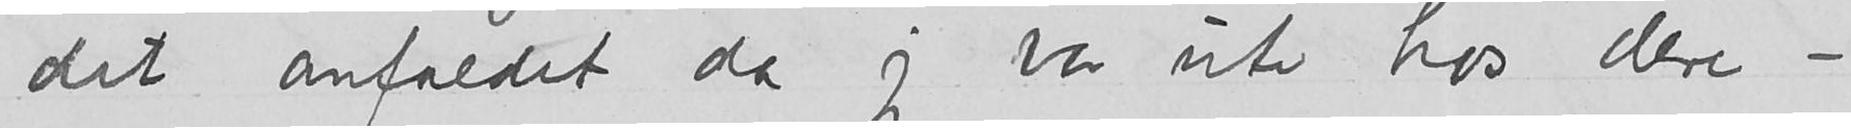det anfaldet da jeg var ute hos dere –.
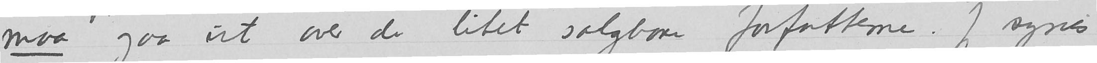maa gaa ut over de litet salgbare forfatterne. Jeg synes
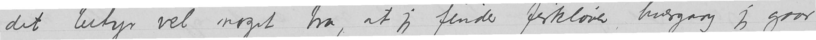det betyr vel noget bra, at jeg finder firkløver hvergang jeg gaar
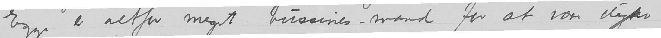Egge er altfor meget bussines-mand for at være digter.
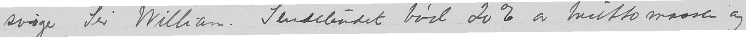sviger Sir William. Sendebudet bød 20% av bruttomassen og
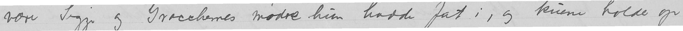være Sigge og Gracchernes mødre hun hadde fat i, og kuene holder op
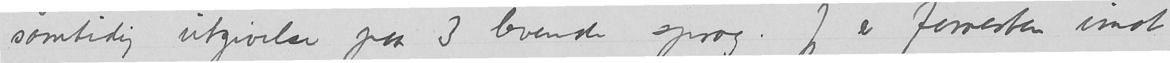samtidig utgivelse paa 3 levende sprog. Jeg er forresten imot
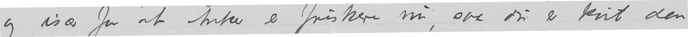og især for at Anker er friskere nu, saa du er kvit
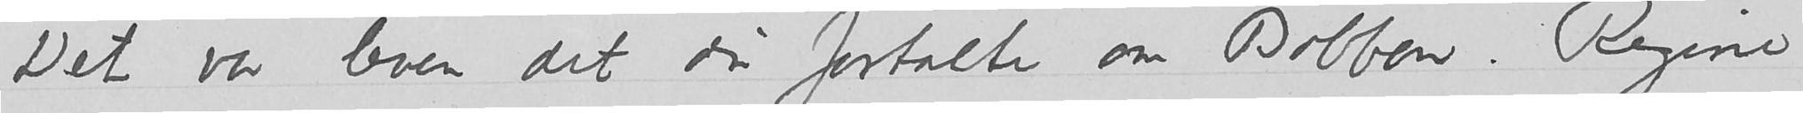Det var leven det du fortalte om Barbra. Regine
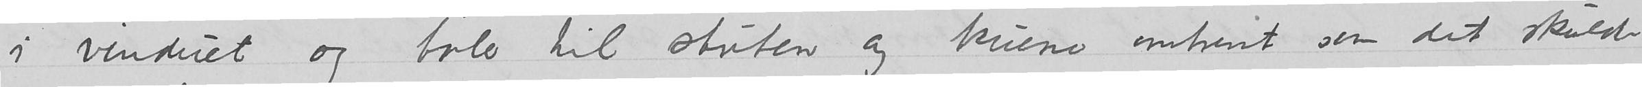i vinduet og taler til stuten og kuerne omtrent som det skulde
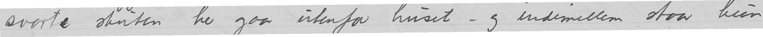svarte stuten her gaar utenfor huset – og indimellem staar hun
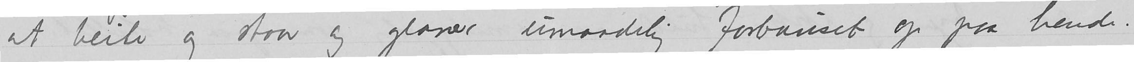at beite og staar og glaner umaadelig forbauset op paa hende.
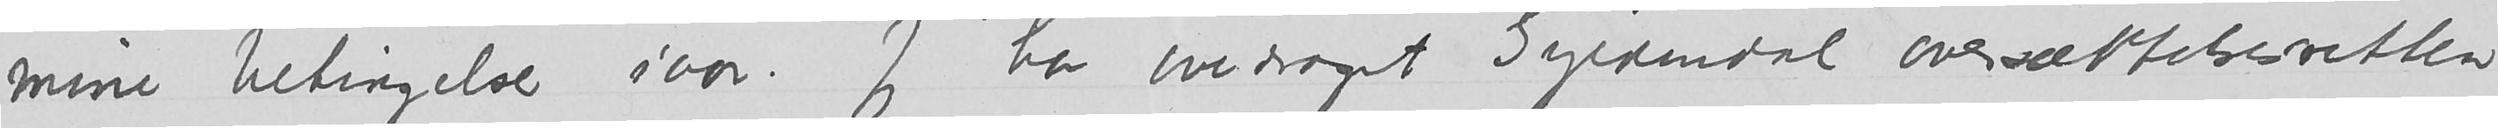mine betingelser iaar. Jeg har overdraget Gyldendal oversættelsesretten
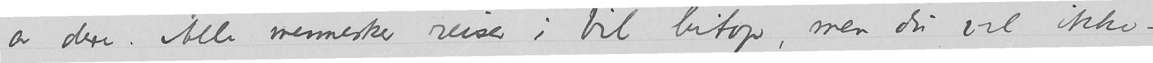av dere. Alle mennesker reiser i bil hitop, men du vel ikke –?
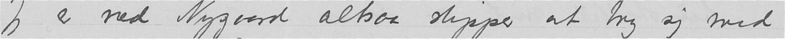Jeg er ræd Nygaard altsaa slipper at bry sig med
